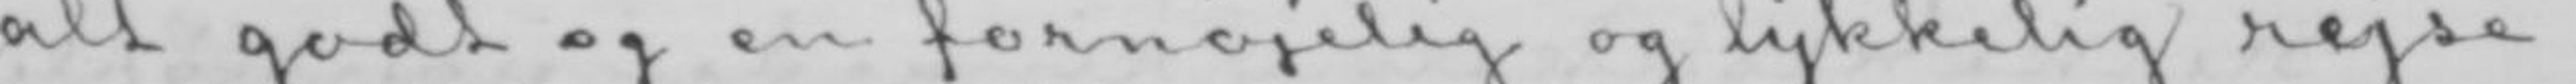alt godt og en fornøjelig og lykkelig rejse.
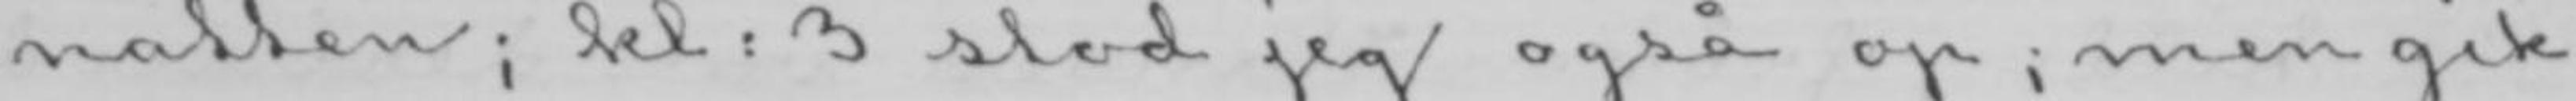natten; kl: 3 stod jeg også op; men gik
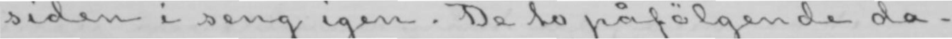siden i seng igen. De to påfølgende da-
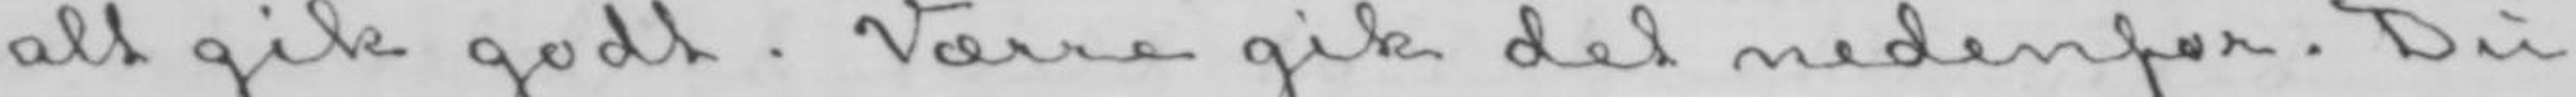alt gik godt. Værre gik det nedenfor. Du
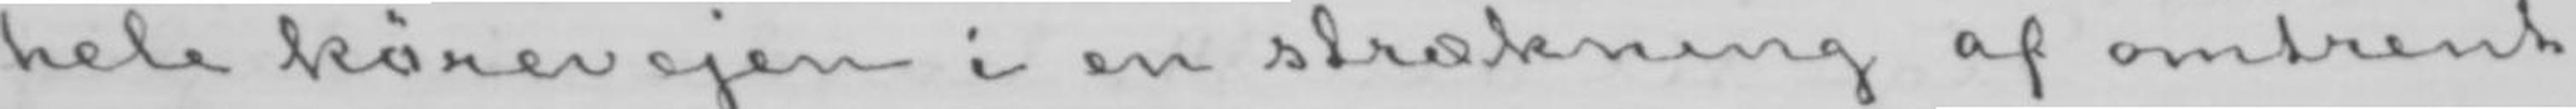hele kørevejen i en strækning af omtrent
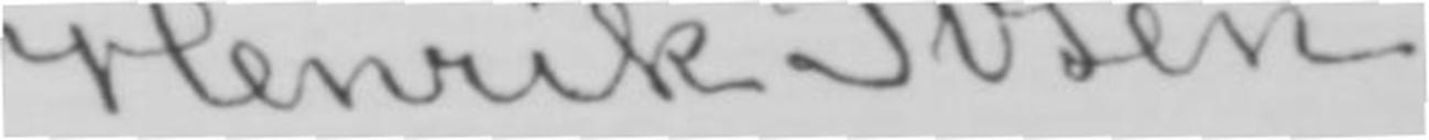Henrik Ibsen.
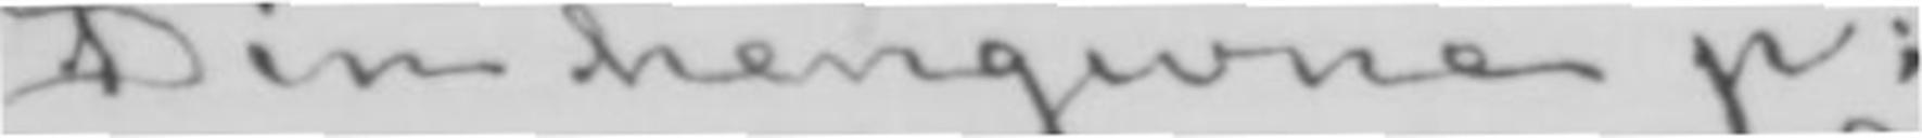Din hengivne p:
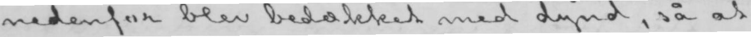nedenfor blev bedækket med dynd, så at
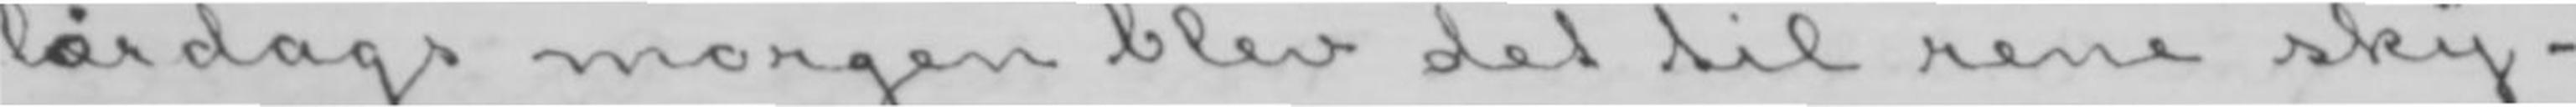lørdags morgen blev det til rene sky-
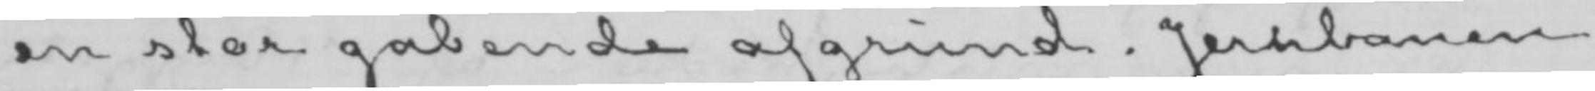en stor gabende afgrund. Jernbanen
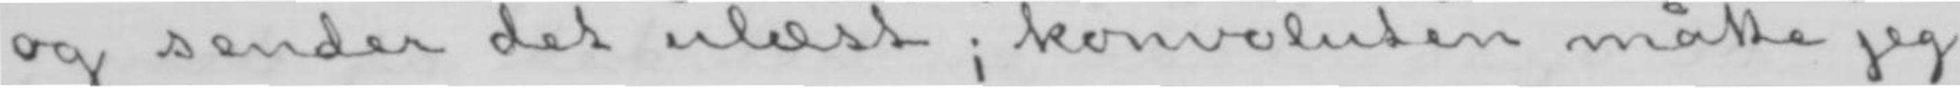og sender det ulæst; konvoluten måtte jeg
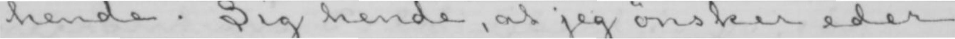hende. Sig hende, at jeg ønsker eder
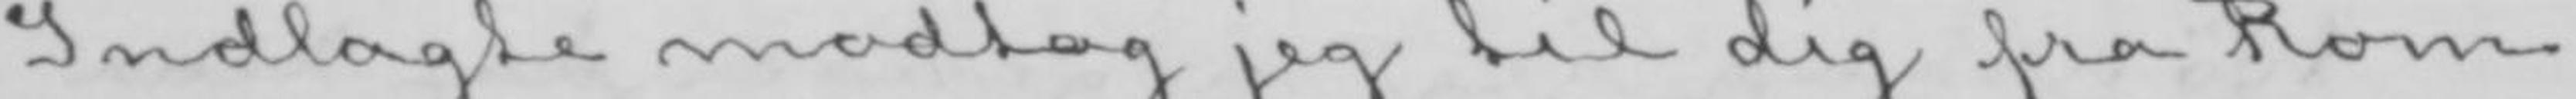Indlagte modtog jeg til dig fra Rom
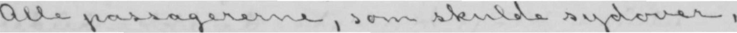Alle passagererne, som skulde sydover,
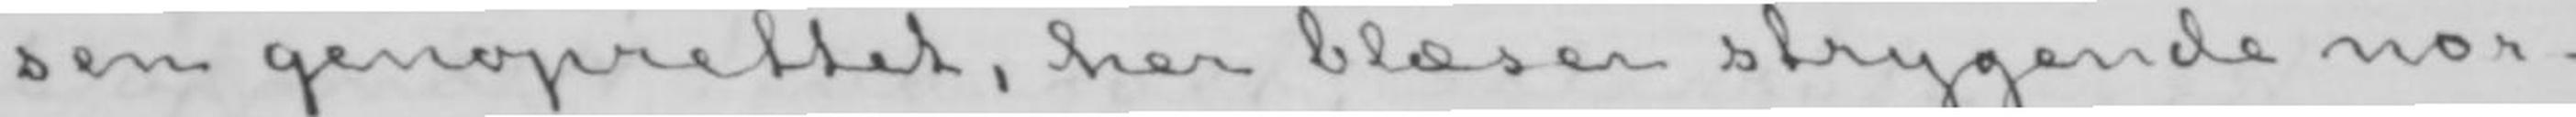sen genoprettet, her blæser strygende nor-
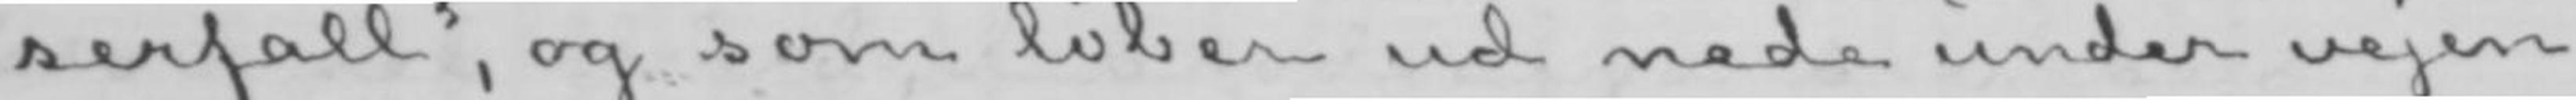serfall», og som løber ud nede under vejen
